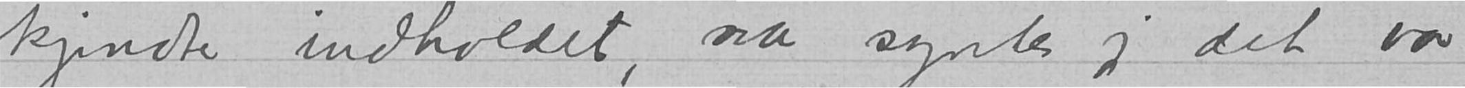kjendte indholdet, saa syntes jeg det var
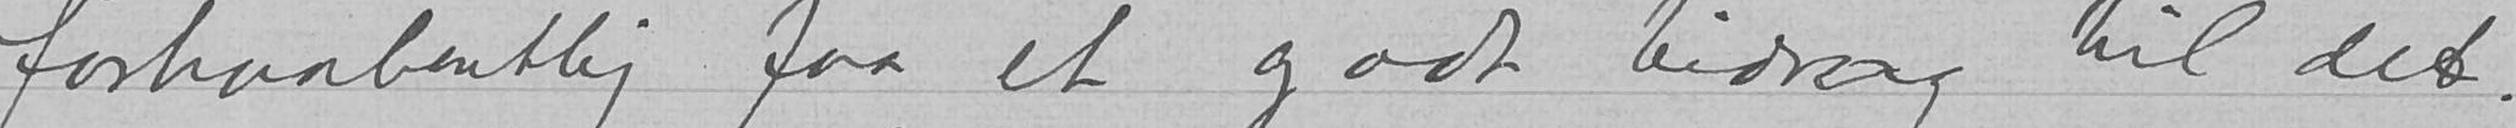forhaabentlig faa et godt bidrag til deg.
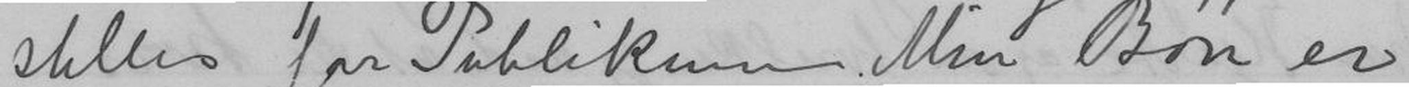stilles for Publikum. Min Bøn er
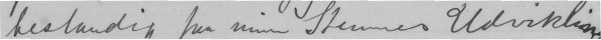bestandig for min Stemmes Udvikling
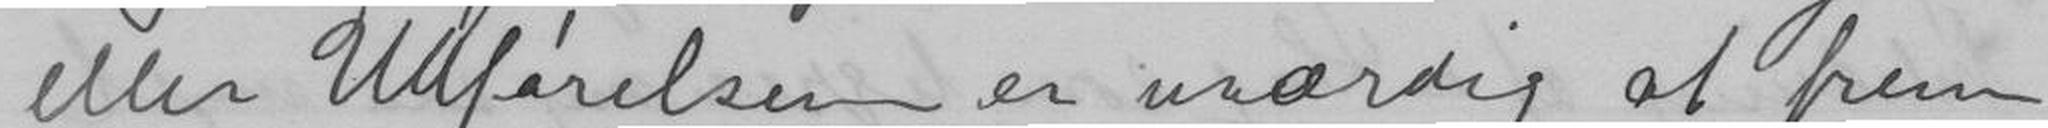eller Udførelsen er uværdig at frem-
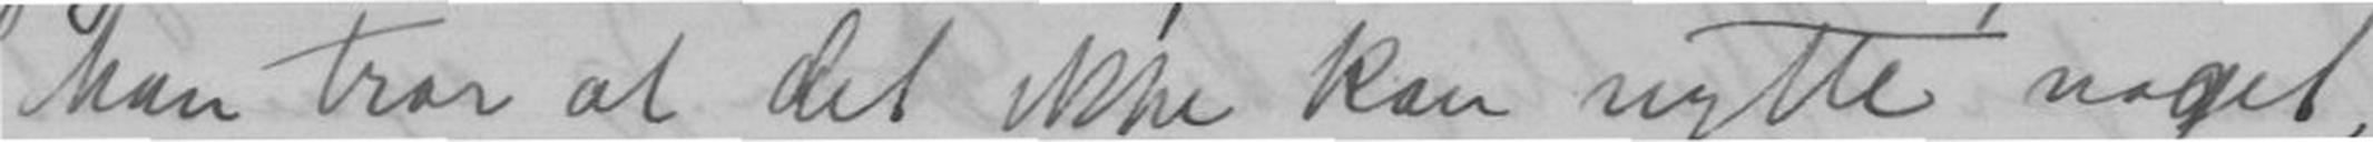han tror at det ikke kan nytte noget;
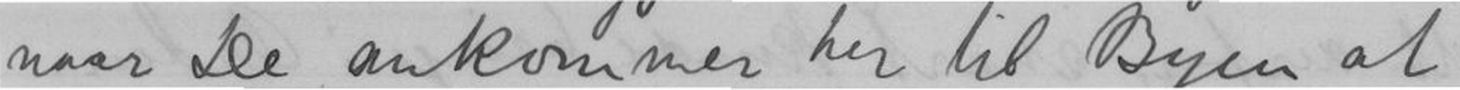naar De ankommer her til Byen at
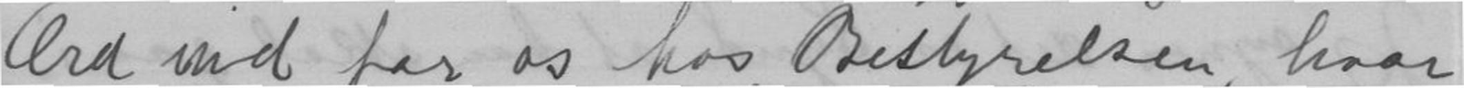Ord ind for os hos Bestyrelsen, hvor
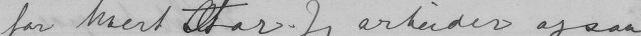for hvert Aar. Jeg arbeider ogsaa
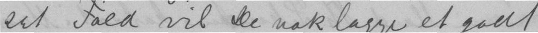sat Fald vil De nok lægge et godt
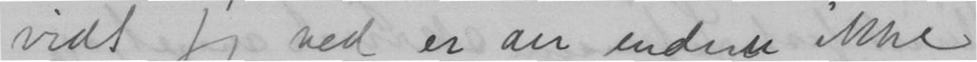vidt jeg ved er der endnu ikke
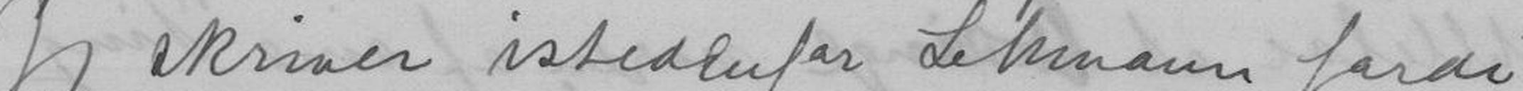Jeg skriver istendenfor Lehmann fordi
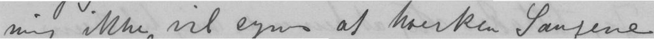mig ikke vil synes at hverken Sangene
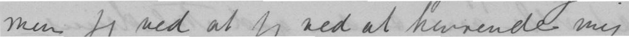men jeg ved at jeg ved at henvende mig
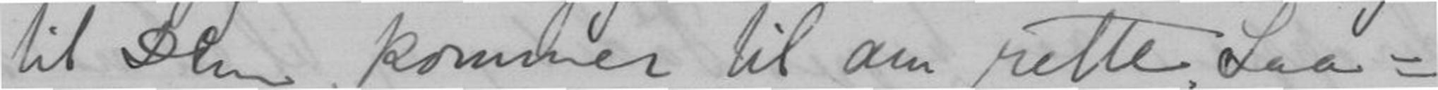til Dem, kommer til den rette; Saa-
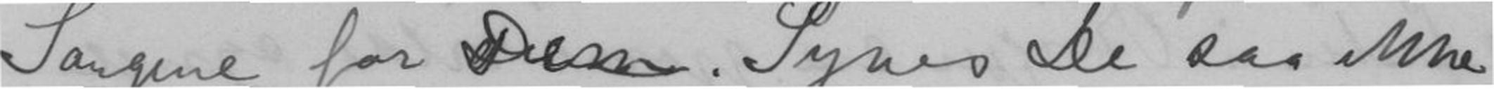Sangene for Dem. Synes De saa ikke
